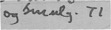og smulg. II
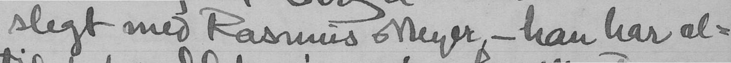slegt med Rasmus Meyer - han har al-
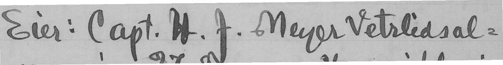Eier: Capt. H.J. Meyer Vetrlidsal-
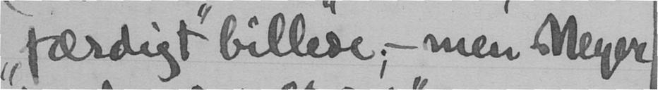"færdigt" billede; - men Meyer
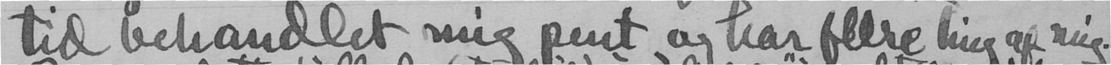tid behandlet mig pent og har flere ting af mig.
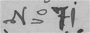No 71
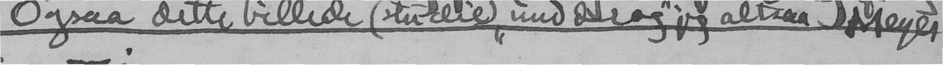Ogsaa dette billede (studie) "unddrog" jeg altsaa Meyer
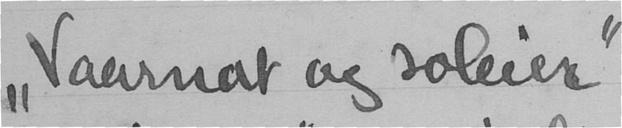"Vaarnat og soleier"
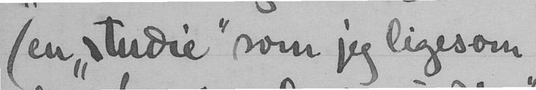(en "studie" som jeg ligesom
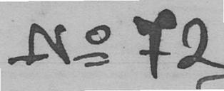N0 72
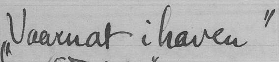"Vaarnat i haven"
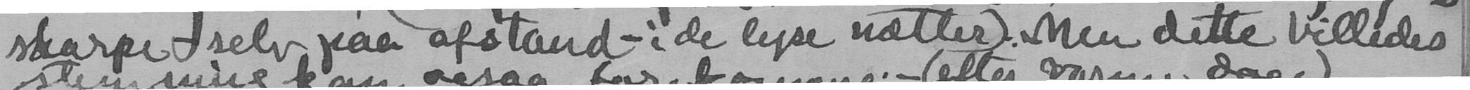skarpe - selv paa afstand - i de lyse nætter). Men dette billedes
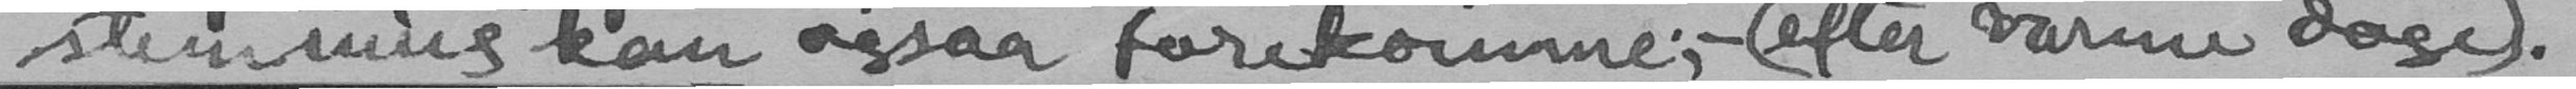stemning ka ogsaa forekomme; - (efter varme dage).
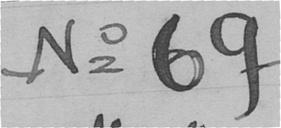No 69
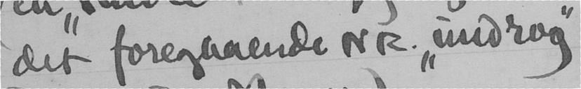det foregaaende NR. "undrog"
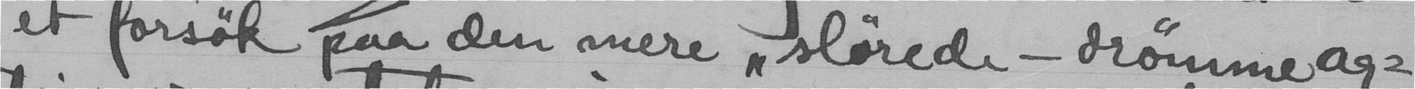et forsøk paa den mere "slørede" - drømme ag-
